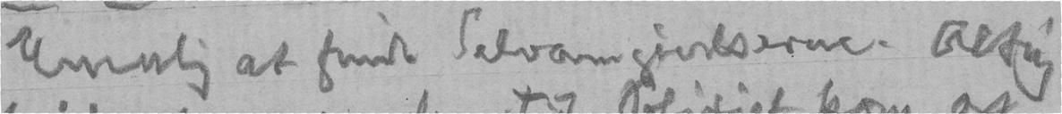Umulig at finde Selvangivelserne. Alting
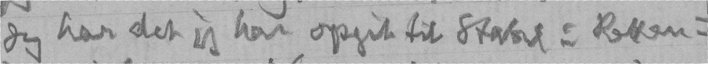Jeg har det jeg har opgit til Stabel i Retten:
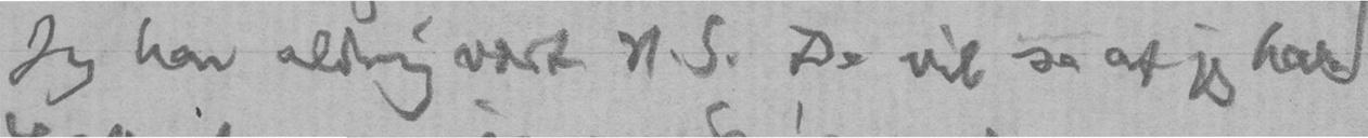Jeg har aldrig vært N.S. De vil sa at jeg har
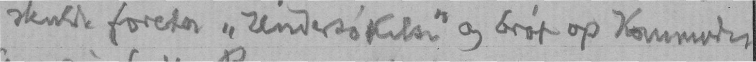skulde foreta "Undersøkelse" og brøt op Kommoden
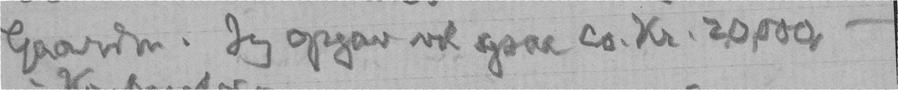Gaarden. Jeg opgav vel ogsaa ca. Kr. 20,000, -
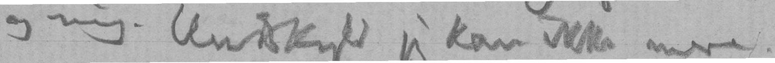og mig. Undskyld jeg kan ikke mere.
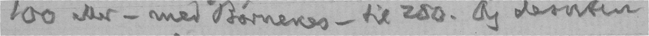100 eller - med Børnenes - til 200. Og desuten
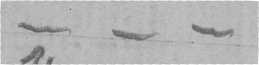- - -
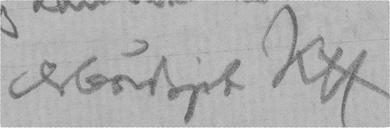Ærbødigst KH
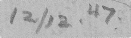12/12. 47.
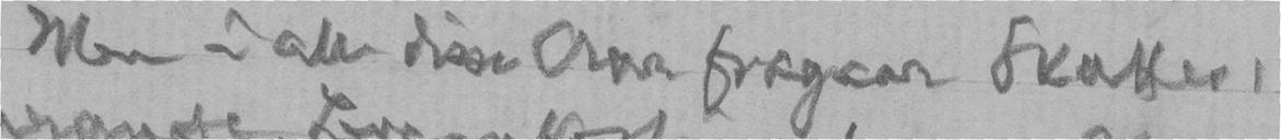Men i alle disse Aar fragaar Skatter,
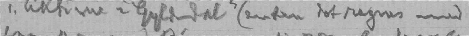"Aktiene i Gyldendal (enten det regnes med
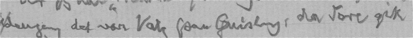dengang det var Valg paa Quisling, da Tore gik
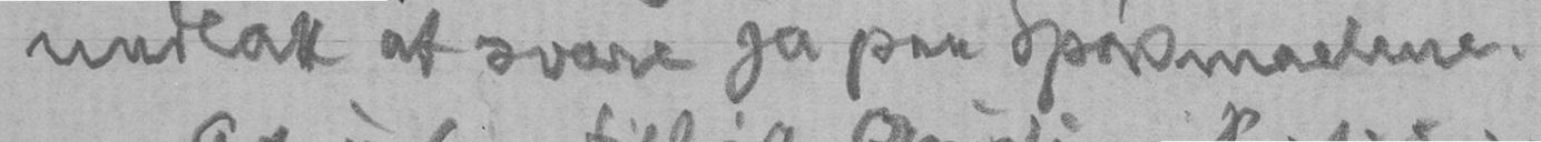undlatt at svare ja paa Spørsmaalene.
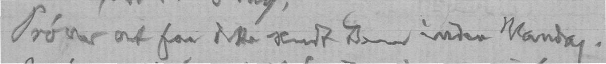Prøver at faa dette sendt Dem inden Mandag.
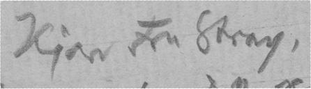Kjære Fru Stray,
